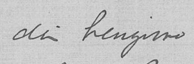din hengivne
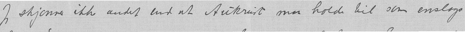Jeg skjønner ikke andet end at Aukrust maa holde til som enslags
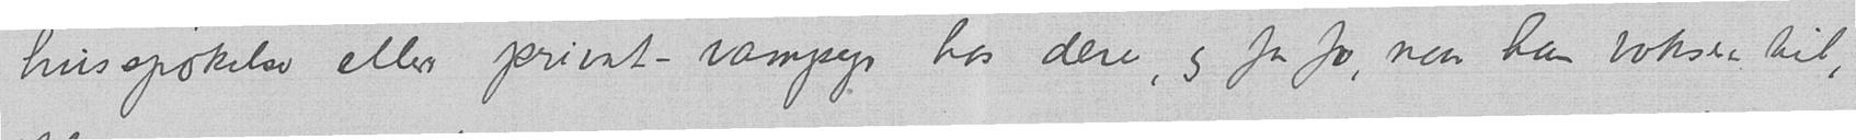husspøkelse eller privat-vampyr hos dere, og for Jo, naar han vokser til,
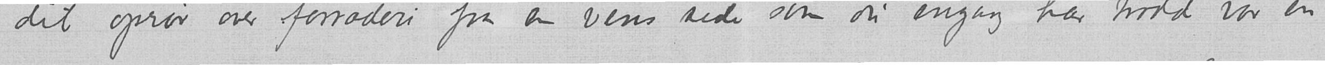dit oprør over forræderi fra en vens side som du engang har trodd var en
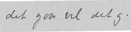det gaar vel det og.
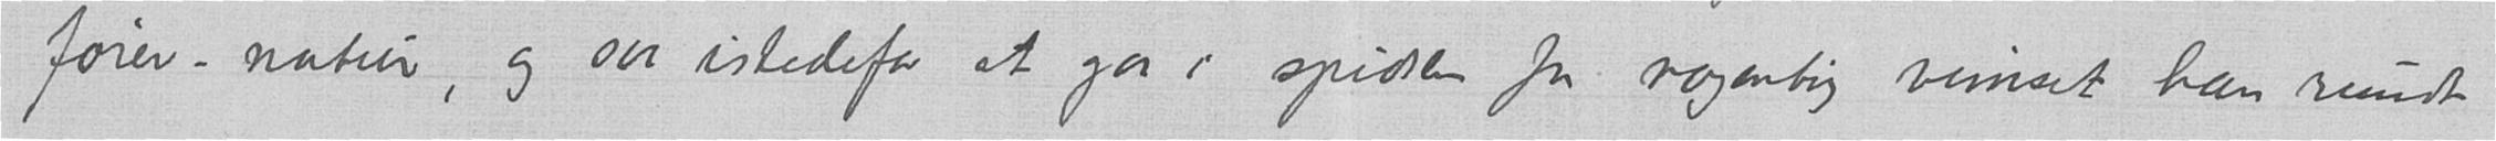fører-natur, og saa istedefor at gaa i spidsen for nogenting vimset han rundt
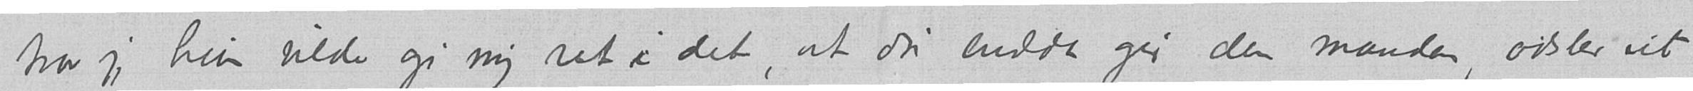tror jeg hun vilde gi mig ret i det at du endda gir den manden, ødsler ut
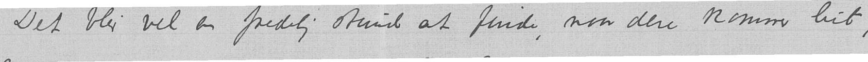Det blir vel en fredelig stund at finde, naar dere kommer hit,
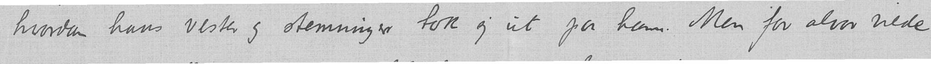hvordan hans vester og stemninger tok sig ut paa ham. Men for alvor vilde
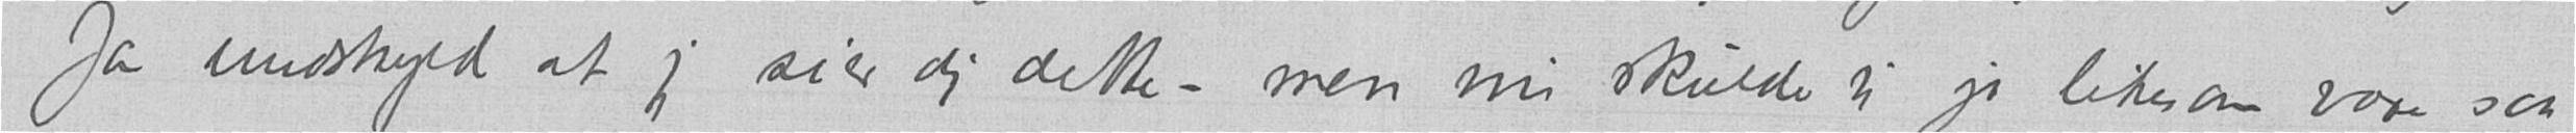Ja undskyld at jeg sier dig dette – men nu skulde vi jo likesom være saa
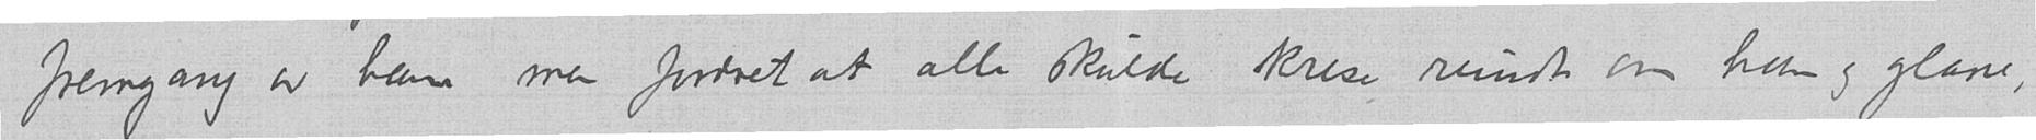fremgang av ham, men fordret at alle skulde kruse rundt om ham og glane,
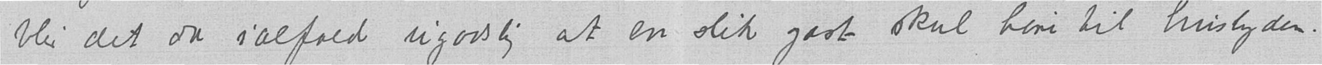blir det da ialfald ugodslig at en slik gæst skal høre til huslyden.
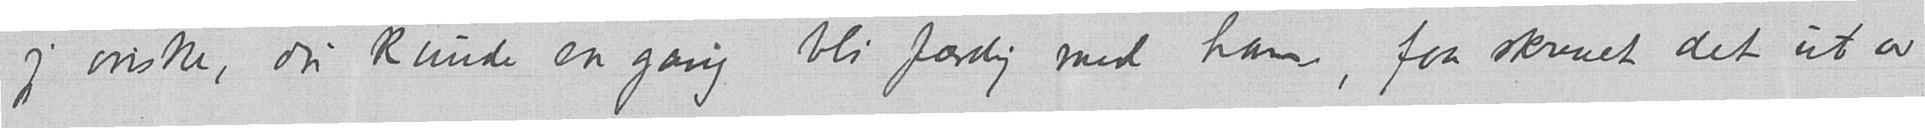jeg ønske, du kunde en gang bli færdig med ham, faa skrevet det ut av
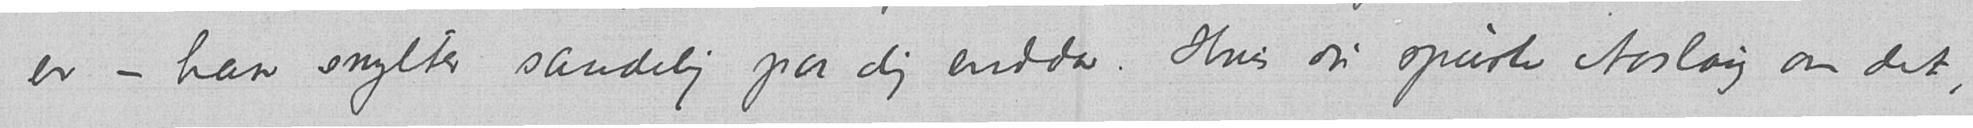er – han snylter sandelig paa dig endda. Hvis du spurte Aaslaug om det,
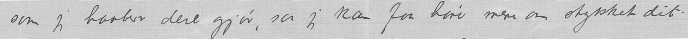som jeg haaber dere gjør, saa jeg kan faa høre mere om stykket dit.
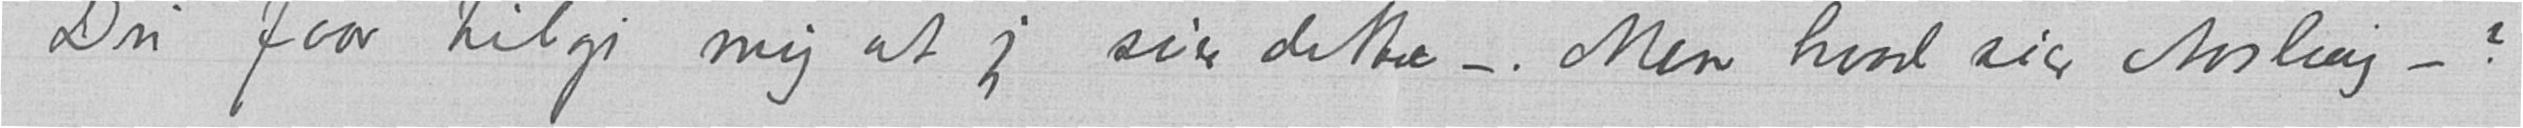Du faar tilgi mig at jeg sier dette –. Men hvad sier Aaslaug –?
